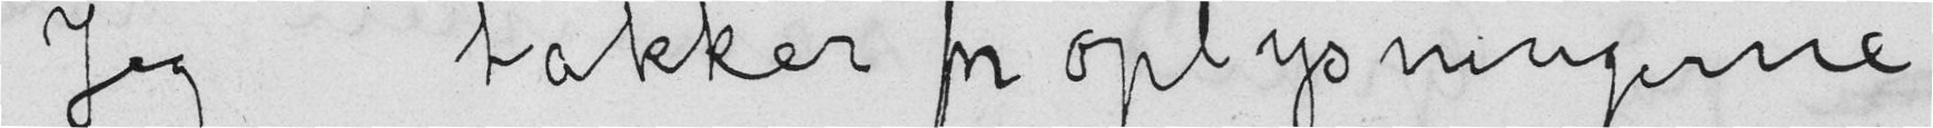Jeg takker for oplysningerne
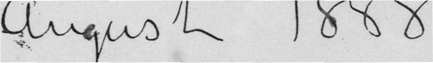August 1888
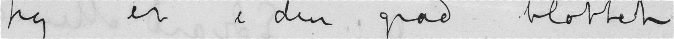Jeg er i den grad blottet
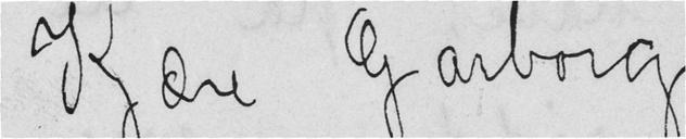Kjære Garborg!
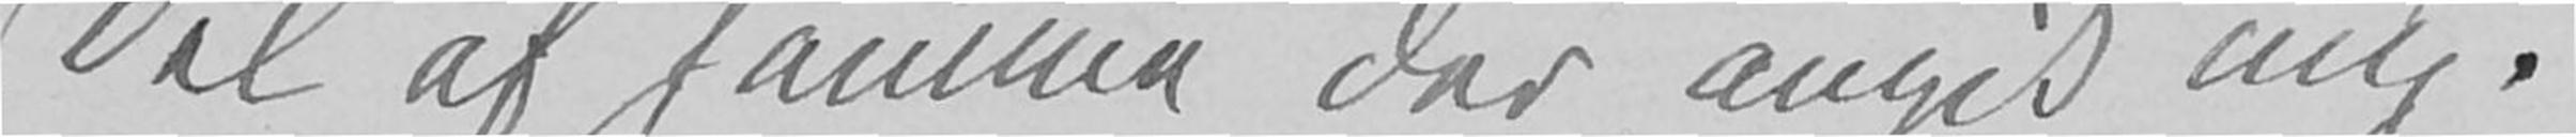Del af samme der angik mig.
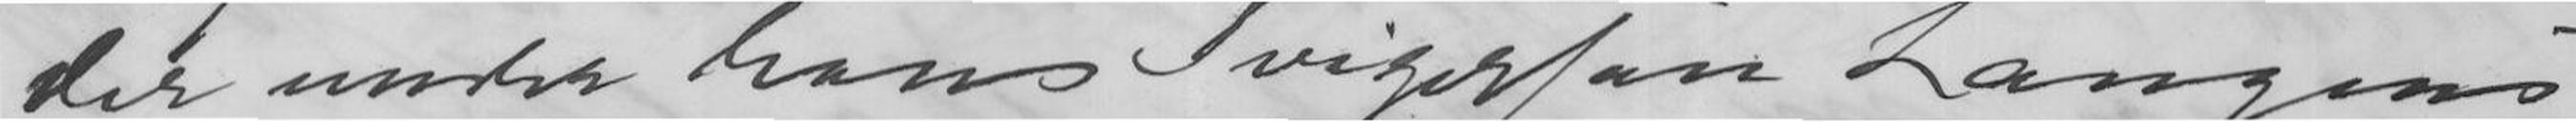der under hans Svigerson Langens
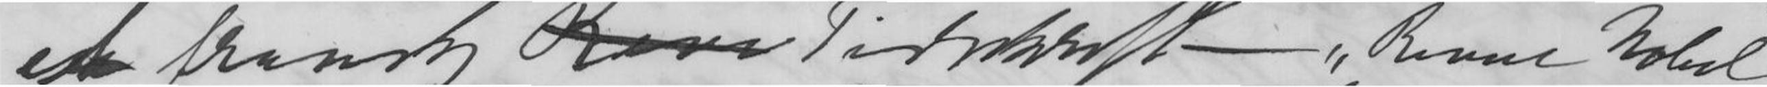et fransk Reve Tidskrift Revue Nobel
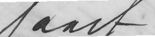faaet
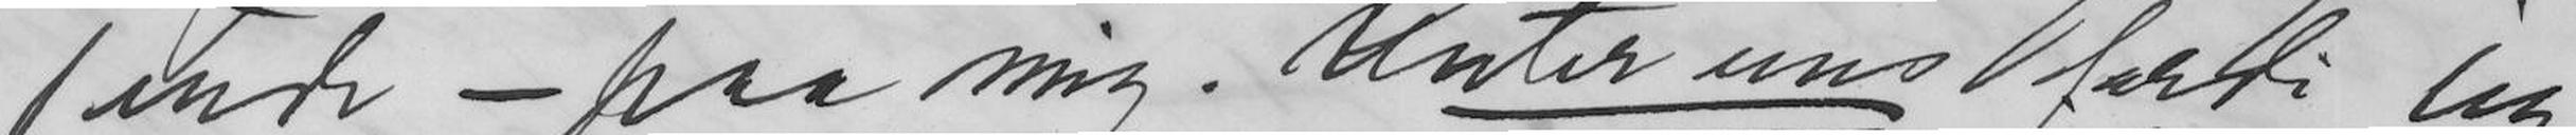sende - paa mig. Unter uns fordi jeg
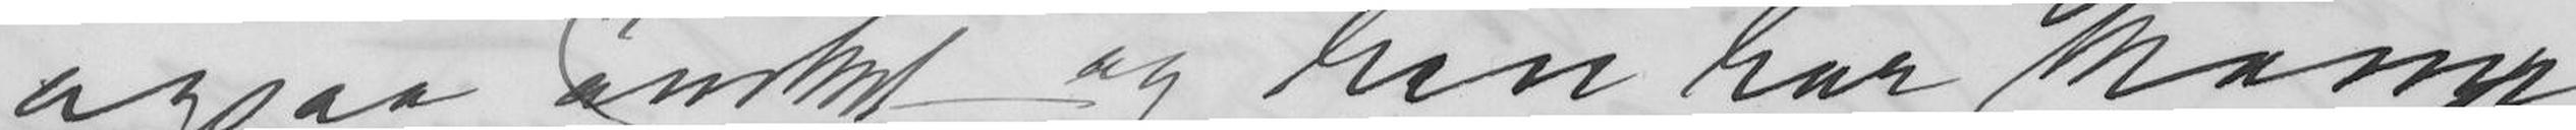ogsaa ønsket og han har komp-
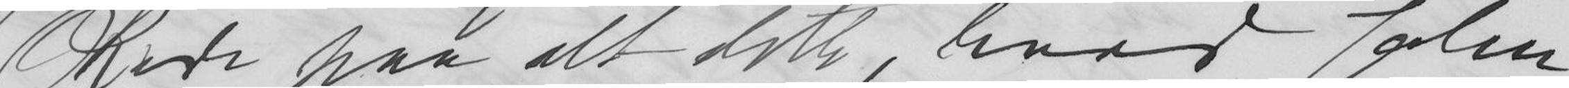Rede paa alt dette, hvad John
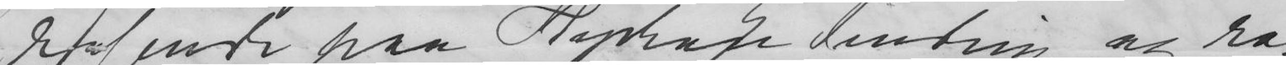rasende paa Stephan Sinding og ra-
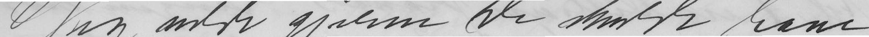Jeg vilde gjerne De skulde have
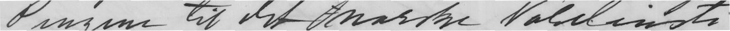Pengene til det norske Nobelinsti-
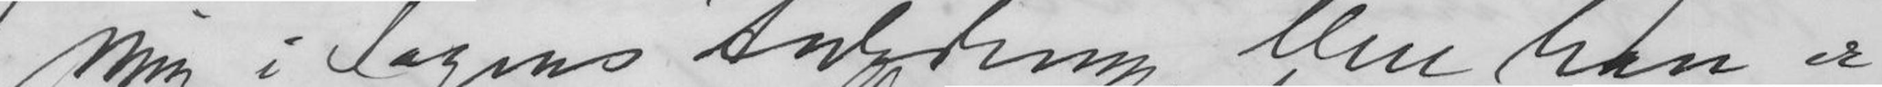mig i Sagens Anledning. Men han er
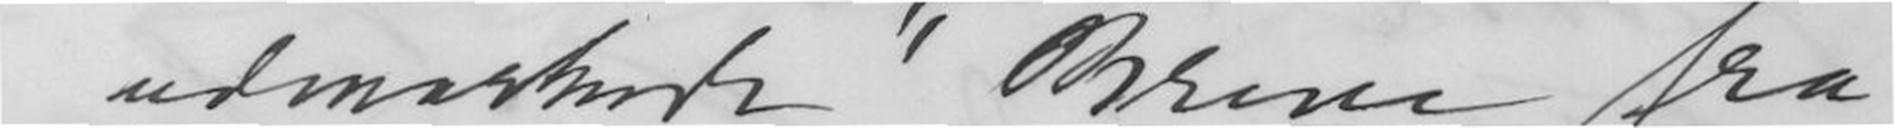"udmærkede" Breve fra
